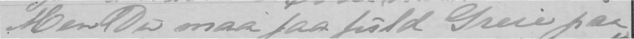Men Du maa faa fuld Greie paa
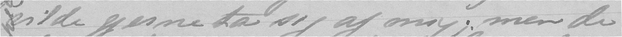vilde gjerne ta sig af mig; men de
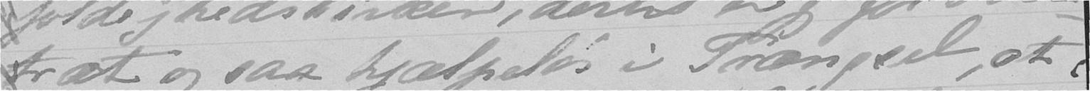træt og saa hjælpeløs i Trængsel, at
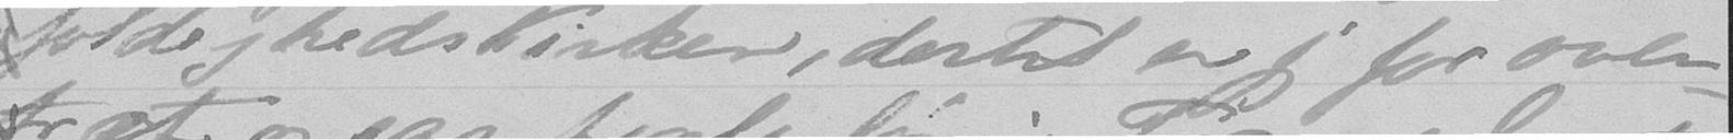foldighedskirken, dertil er jeg for over-
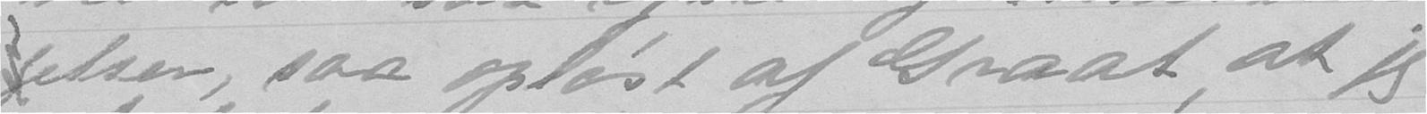gelser, saa opløst af Graat, at jeg
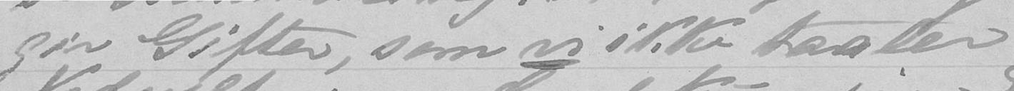gir Gifter, som vi ikke taaler.
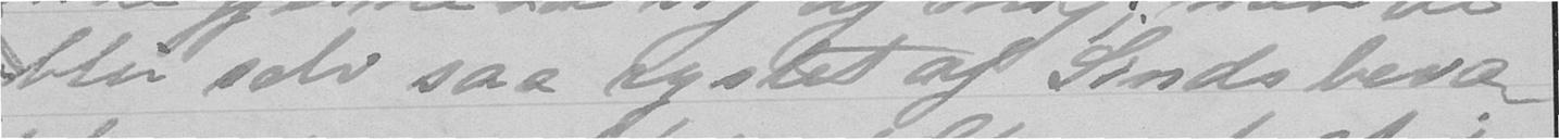blir selv saa rystet af Sindsbevæ-
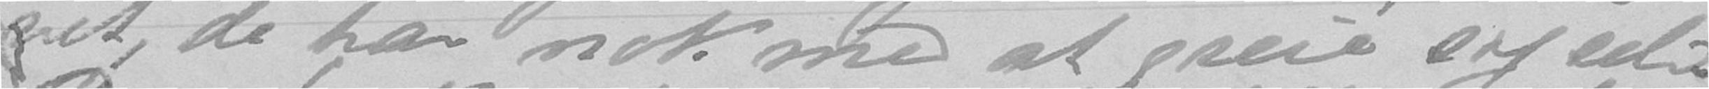vet, de har nok med at greie sig selv.
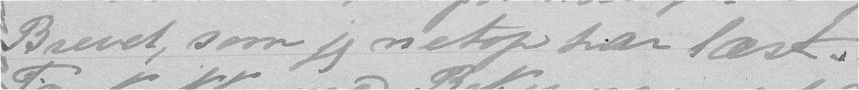Brevet, som jeg netop har læst.
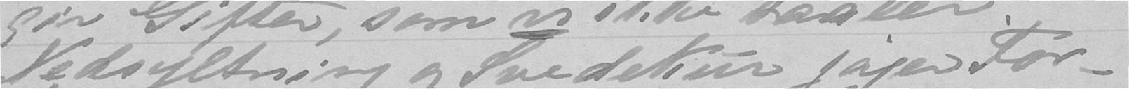Nedsyltning og Svedekur jager For-
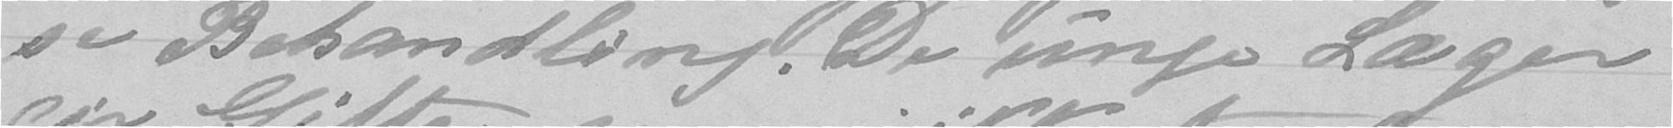se Behandling. De unge Læger
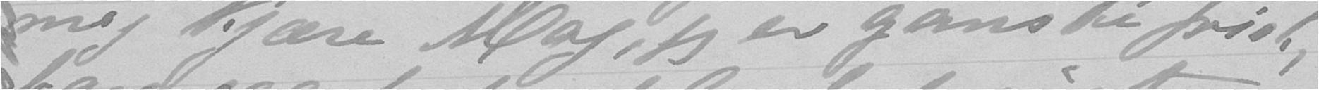mig Kjære Mag, jeg er ganske frisk,
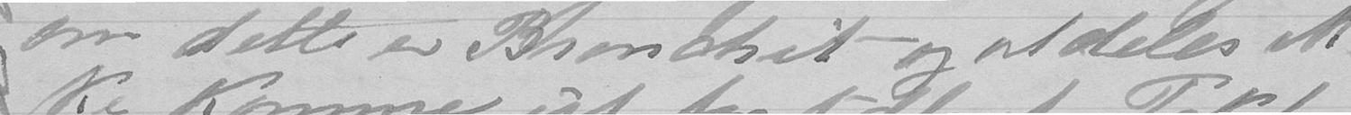om dette er Bronchit og aldeles ik-
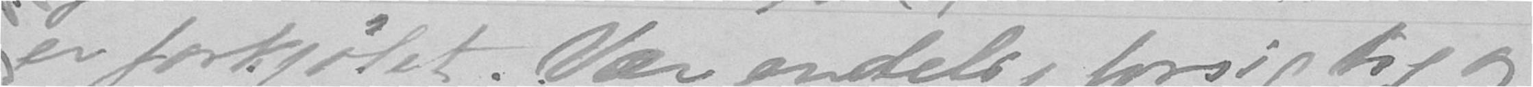er forkjølet. Vær endelig forsigtig og
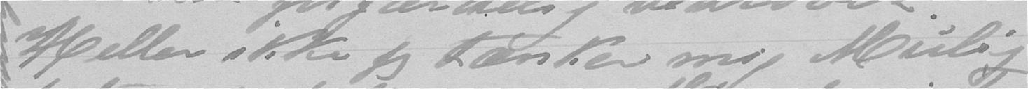Heller ikke jeg tænker mig Mulig-
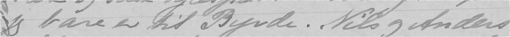jeg bare er til Byrde. Nils og Anders
